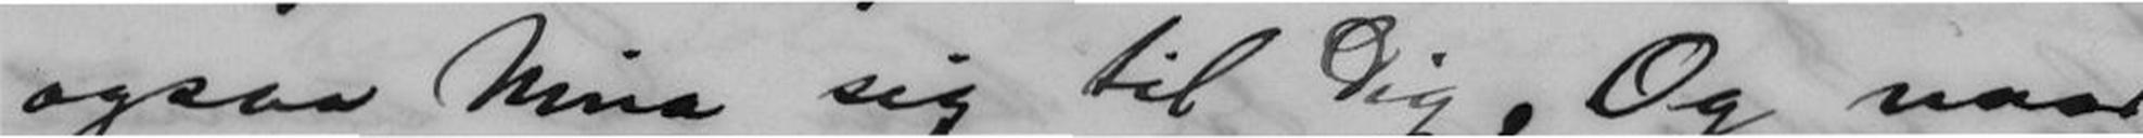ogsaa Nina sig til Dig. Og naar
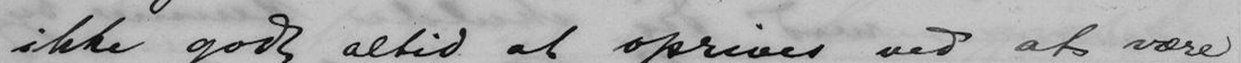ikke godt altid at oprives ved at være
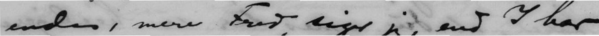ende, mere Fred, siger jeg, end I har
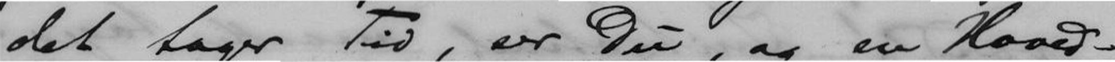det tager Tid, ser Du, og en Hoved-
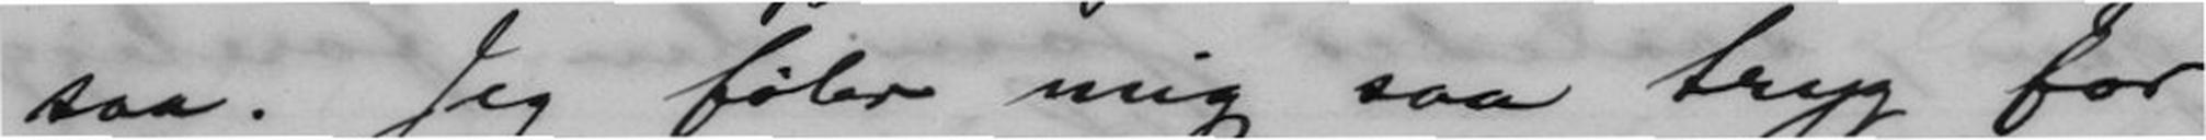saa. Jeg føler mig saa tryg for,
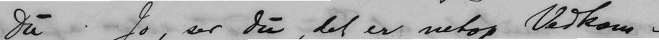Du Jo, ser Du, det er netop Vedkom-
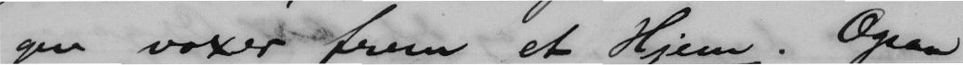gen voxer frem et Hjem. Ogsaa
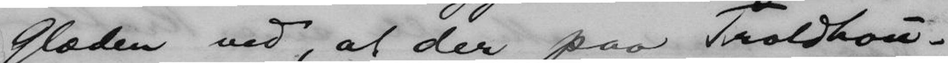Glæden ved, at der paa Troldhou-
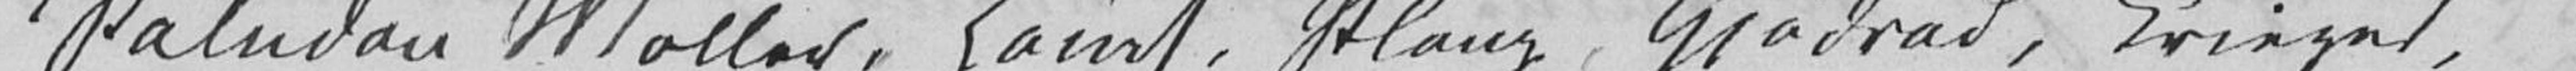Paludan Møller, Hauch, Ploug, Gjødvad, Krieger,
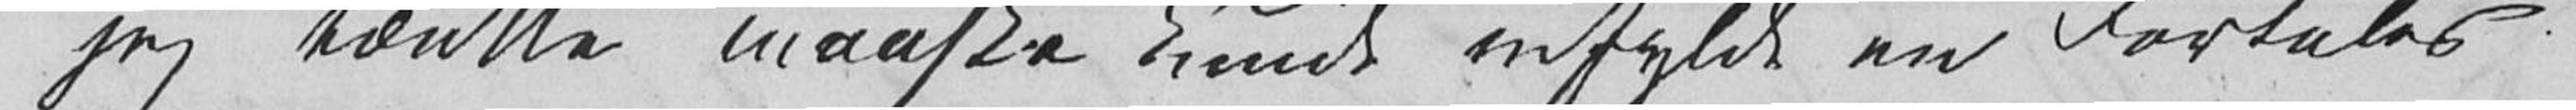jeg tænkte maaske kunde udfylde en Fortales
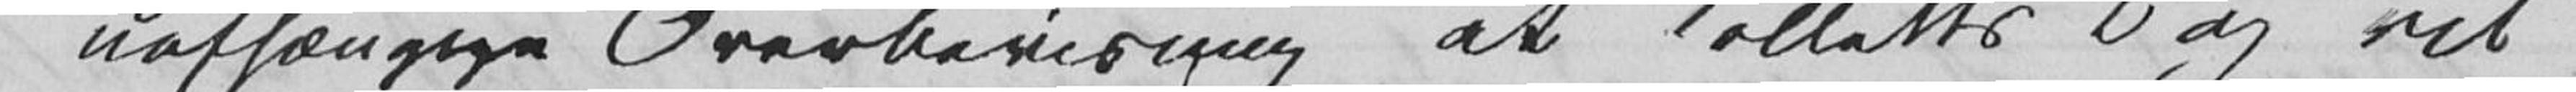uafhængige Overbevisning at Colletts Bog vil
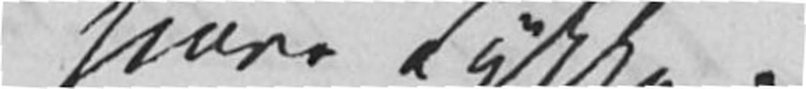gjøre Lykke.
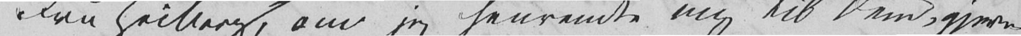Fru Heiberg, om jeg henvendte mig til Dem, gjerne
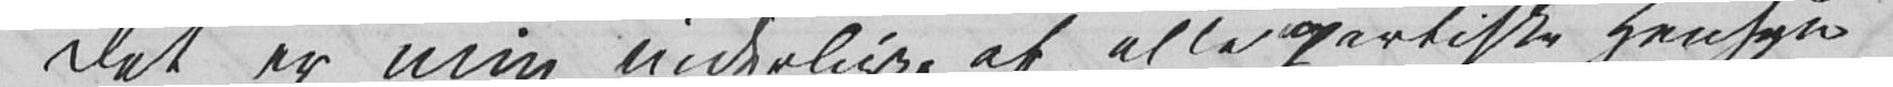Det er min inderlige, af alle partiske Hensyn
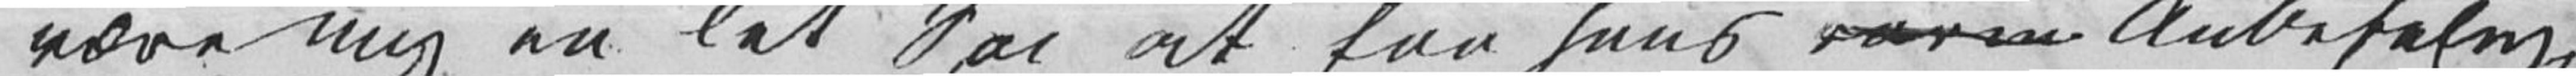være mig en let Sag at faa hans varme Anbefaling,
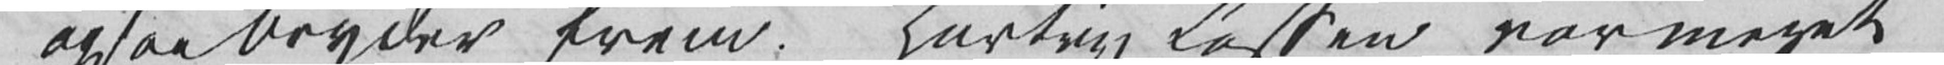ogsaa bryder frem. Hartvig Lassen var meget
